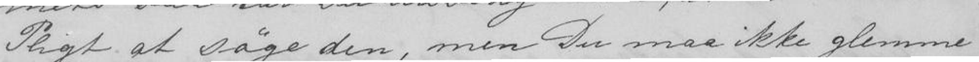Pligt at søge den, men Du maa ikke glemme
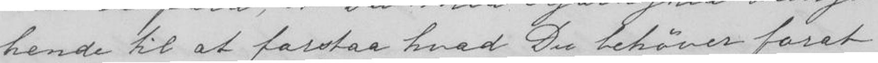hende til at forstaa hvad Du behøver forat
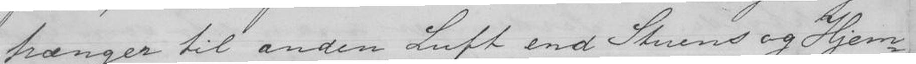trænger til anden Luft end Stuens og Hjem-
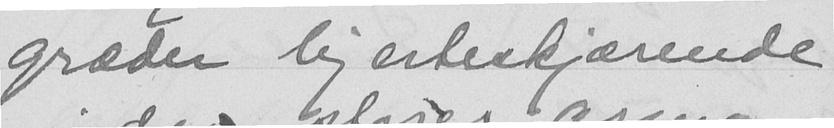græder hjerteskjærende
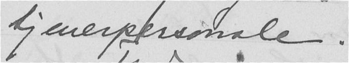tjenerpersonale.
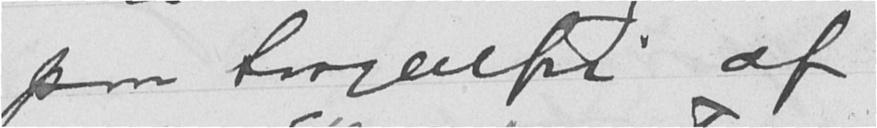paa Sorgenfri af
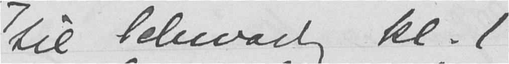til Schwartz kl. 1
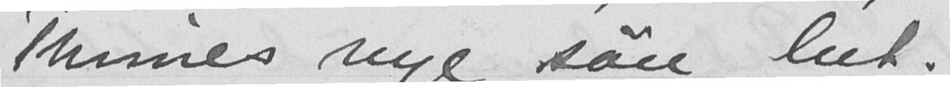Thrines nye søn lnt.
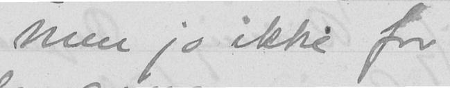Men jo ikke for
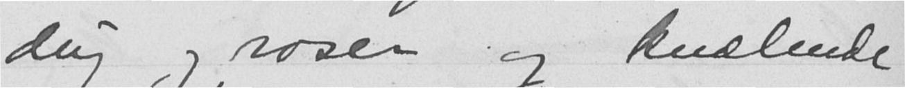dug og roser og knælende
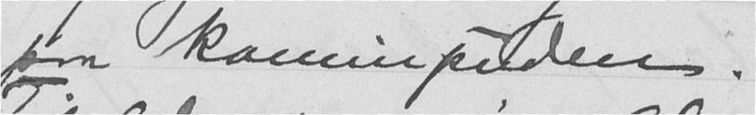paa kaminpuden.
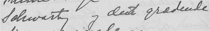Schwartz, og det grædende
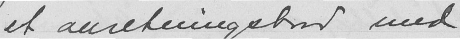et anretningsbord med
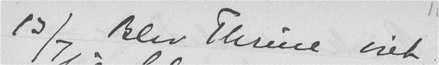13/7 Blev Thrine viet
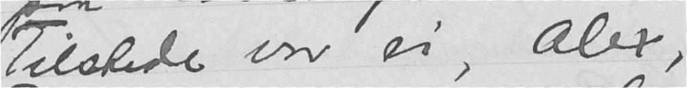Tilstede var vi, Alex,
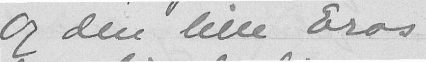Og den lille Eros
